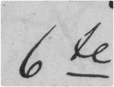6te
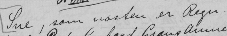Sne, som næsten er Regn.
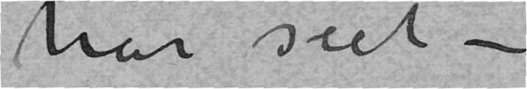har seet –
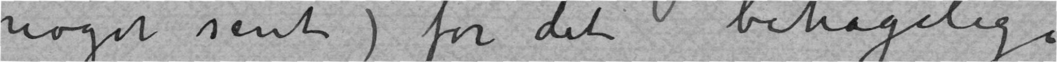noget sent) for det behagelige
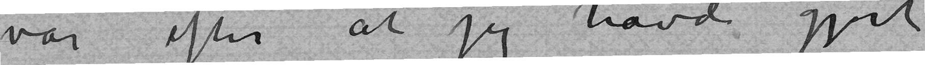var efter at jeg havde gjort
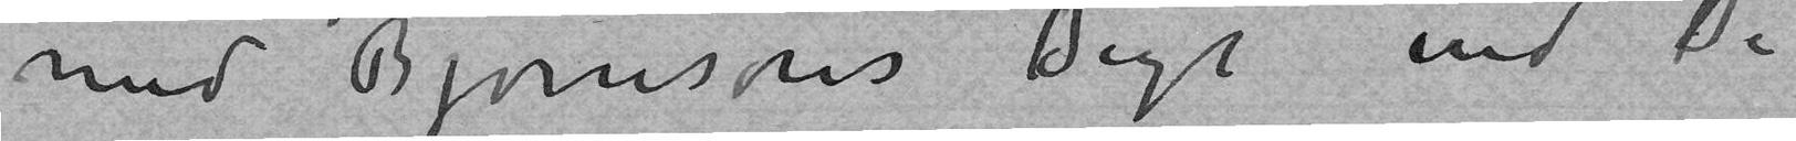med Bjørnsons Digt end De
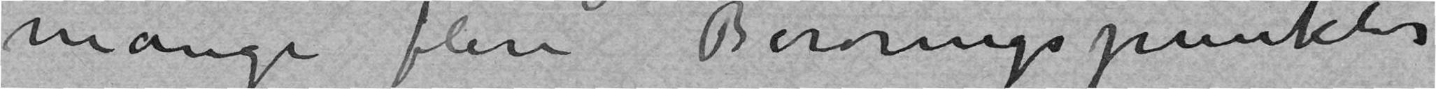mange flere berøringspunkter
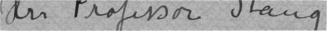Hrr Professor Stang
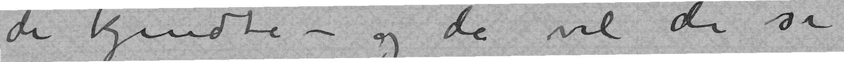de kjendte – og de vil da se
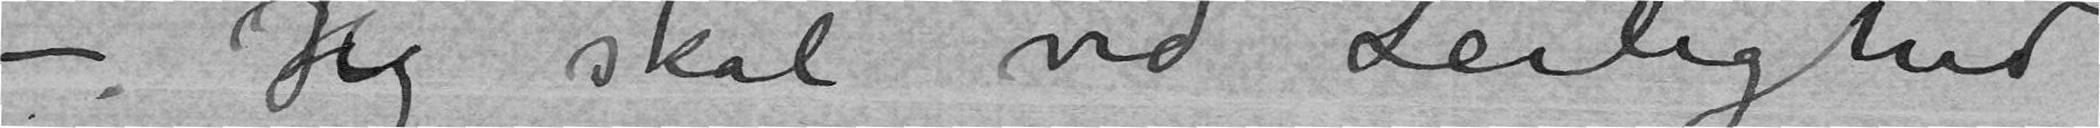– Jeg skal ved Leilighed
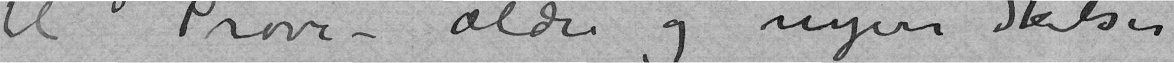til Prøve ældre og nyere Skitser
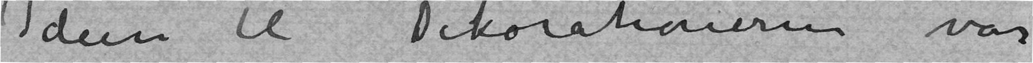Ideen til Dekorasjonerne var
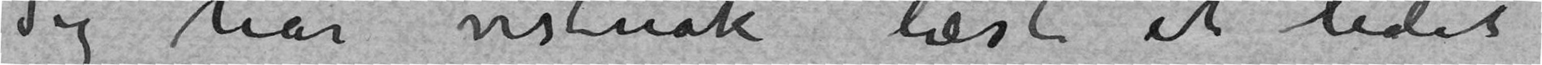Jeg har vistnok læst et lidet
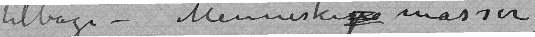tilbage – Menneskepmasser
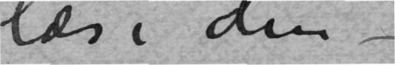læse den –
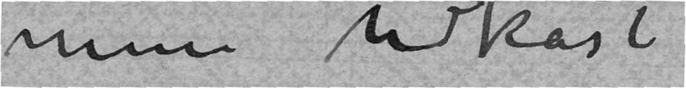mine Udkast
